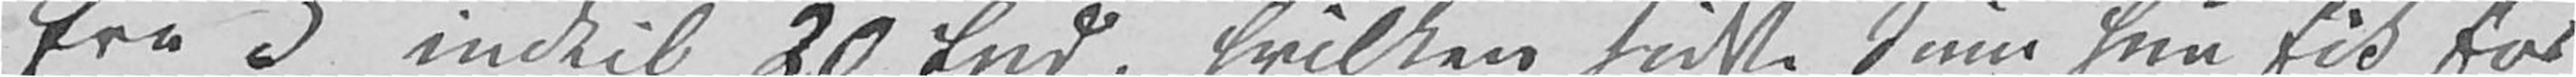fra 5 indtil 20 Spd, hvilken sidste Sum hun fik for
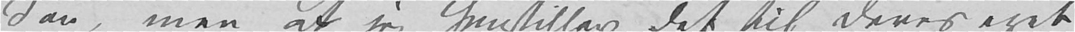Søn, men at jeg henstiller det til Deres eget
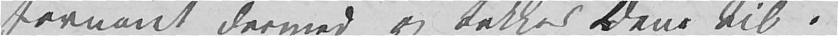fornøiet dermed og takker Dem til.
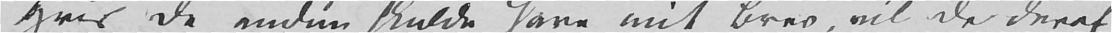Hvis De endnu skulde have mit Brev, vil De deraf
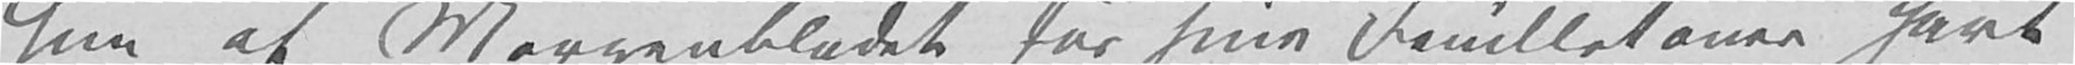hun af Morgenbladet for sine Feuilletoner havt
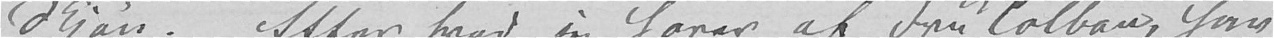Skjøn. Efter hvad jeg hører af Fru Colban, har
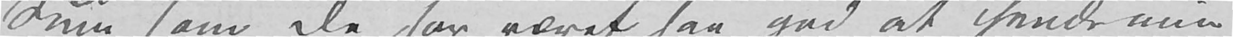Sum som De har været saa god at sende min
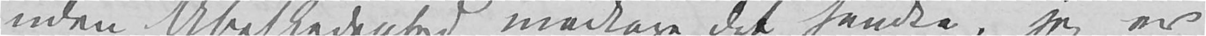uden Ubeskedenhed modtage det sendte, jeg er
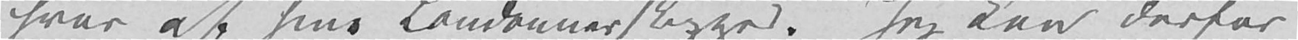hver af sine Londonnerskizzer. Jeg kan derfor
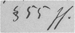§ 55 ff.
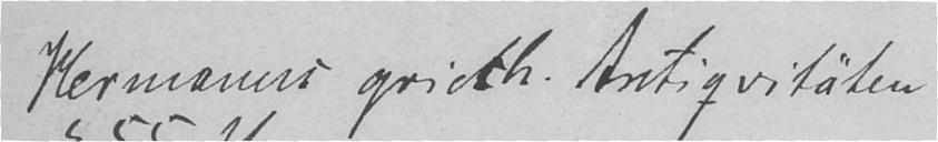Hermanns griech. Antiqvitäten
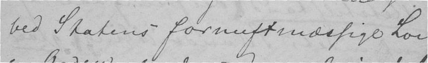ved Statens fornuftmæssige Lov
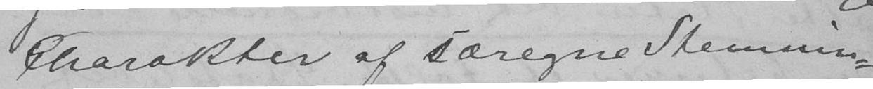Charakter af særegne Stemnin-
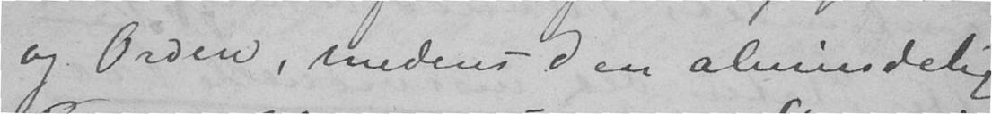og Orden, medens den almindelige
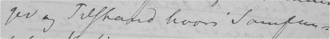ger og Tilstand hvori Samfun-
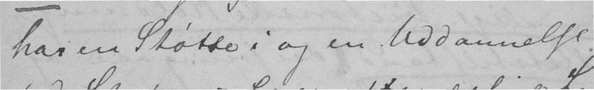har en Støtte i og en Uddannelse
